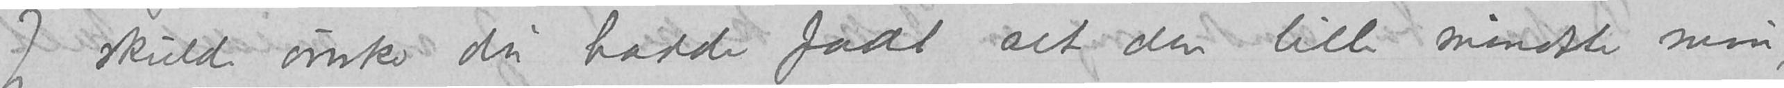Jeg skulde ønske du hadde faat set den lille mindste min,
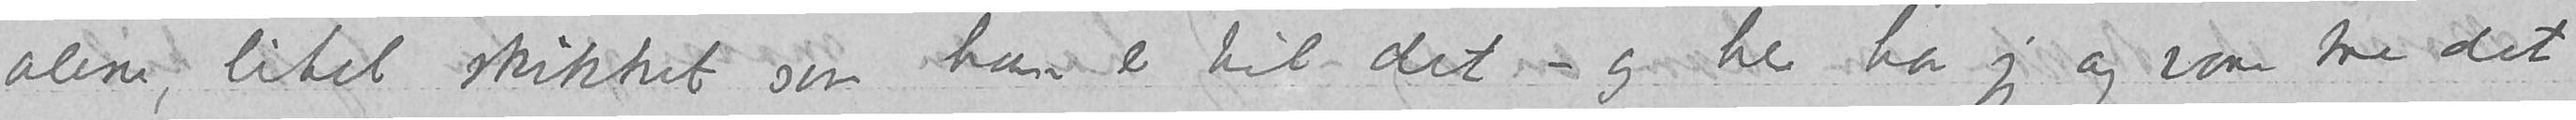alene, litet skikket som han er til det – og her har jeg og vore tre det
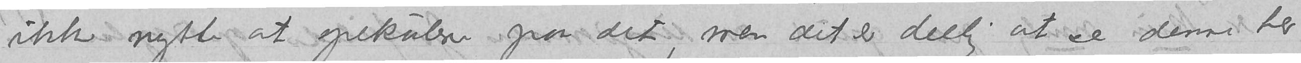ikke nytte at spekulere paa det, men det er deilig at se denne her
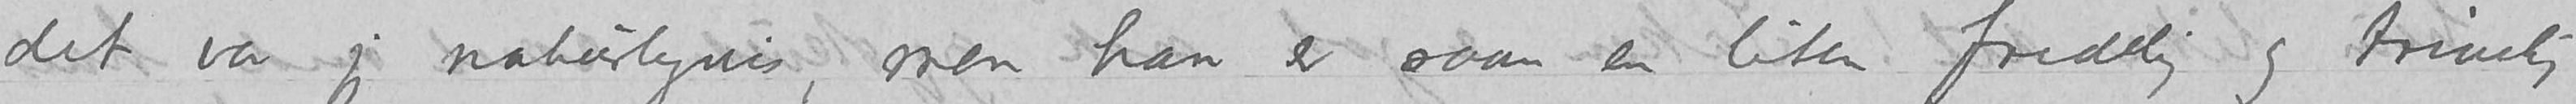det var jeg naturligvis, men han er saan en liten fredelig og trivelig
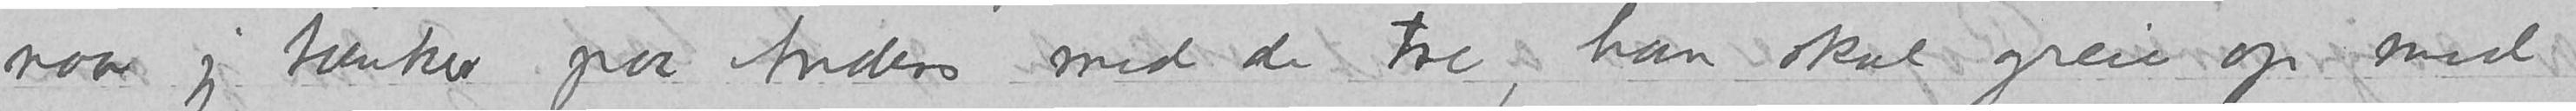naar jeg tænker paa Anders med de tre, han skal greie op med
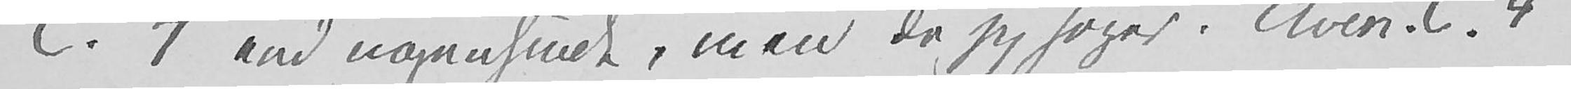C. 4 end nogensinde, men de jeg søger i Aven. C. 4
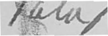tale
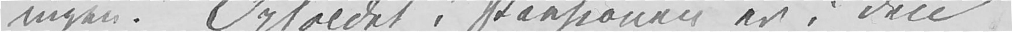ingen. Opholdet i Pensionen er i den
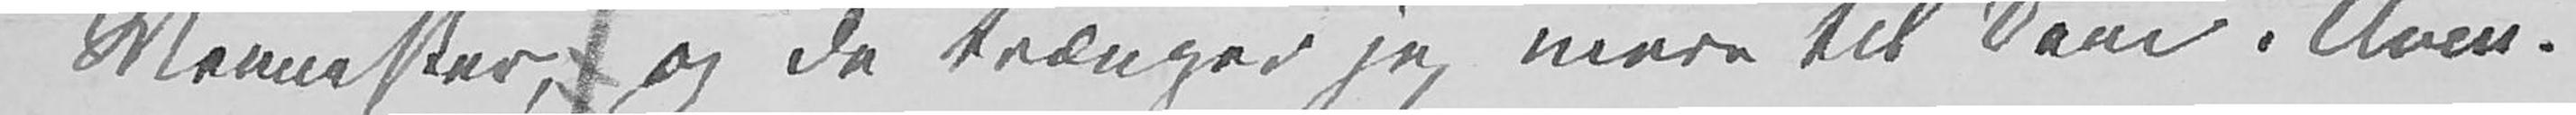Mennesker, og da trænger jeg mere til Dem i Aven.
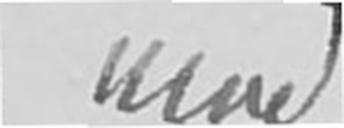med
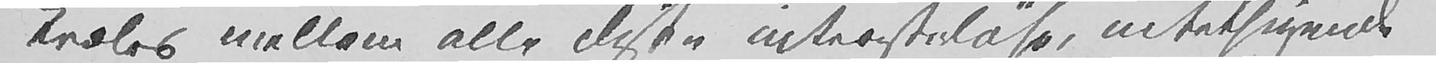kvæles mellem alle disse interesseløse, intetsigende
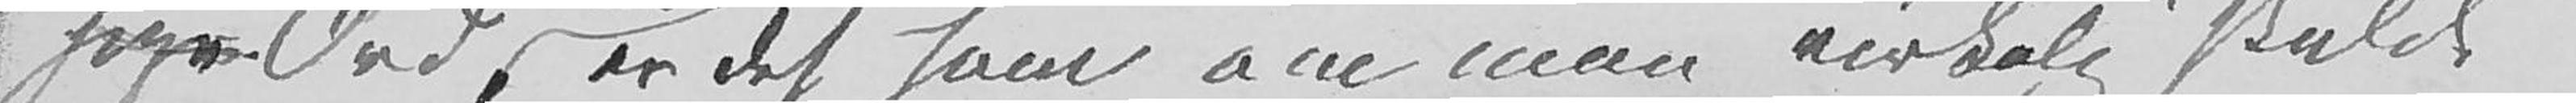sige Ord, er det som om man virkelig skulde
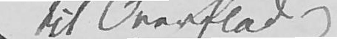til Overflod
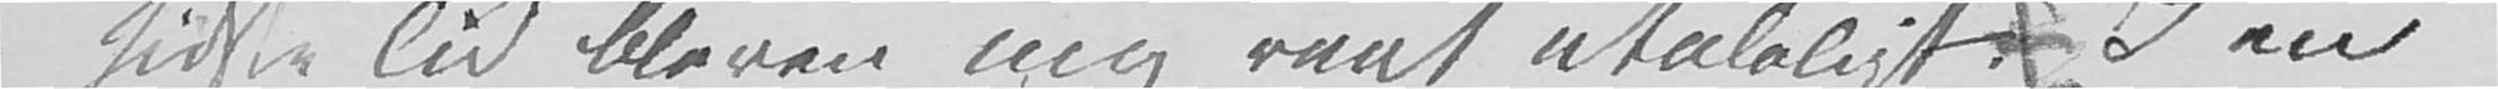sidste Tid bleven mig rent utåleligt. I en
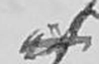et
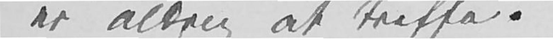er aldrig at treffe.
